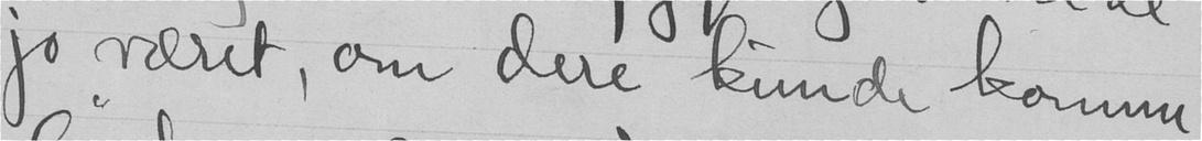jo været, om dere kunde komme
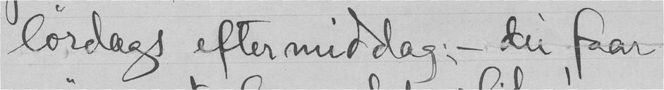lørdags efter middag; - du faar
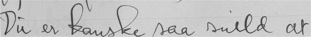Du er kanske saa snild at
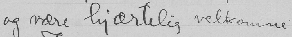og være hjærtelig velkomne
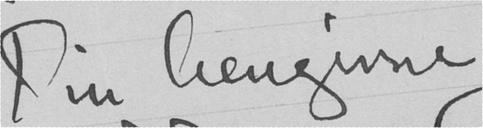Din hengivne
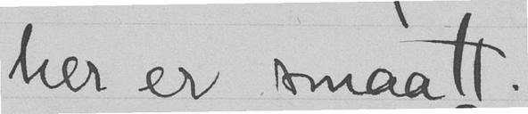her er smaatt.
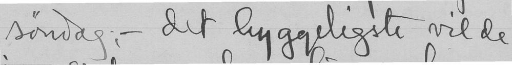søndag; - det hyggeligste vilde
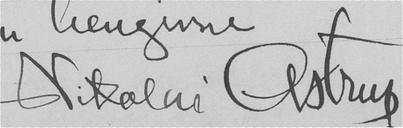Nikolai Astrup
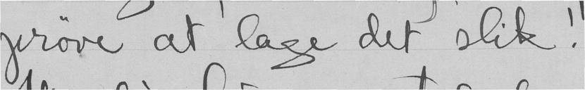prøve at lage det slik!
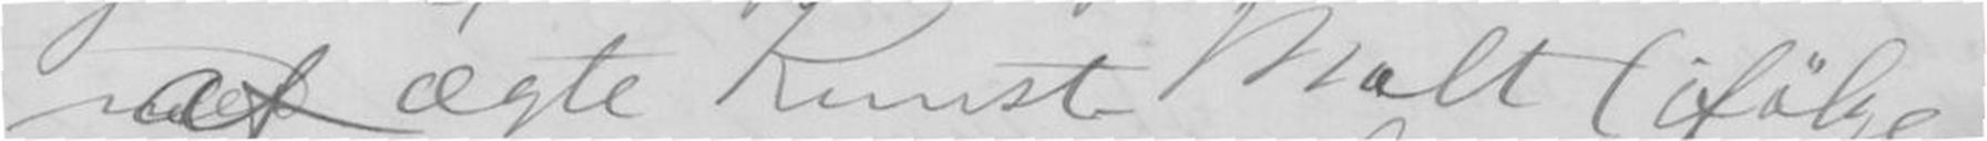af ægte Kunst Malt (ifølge
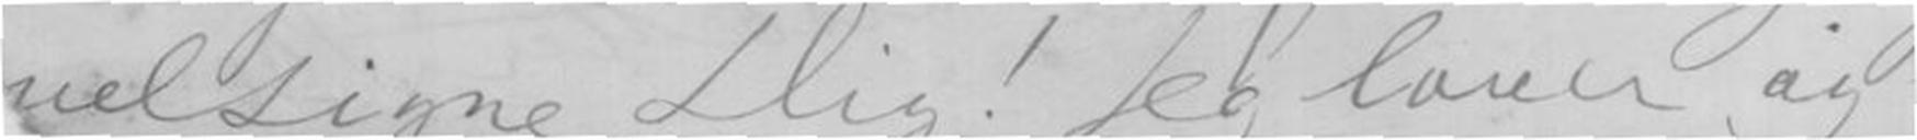velsigne Dig! Jeg lover og
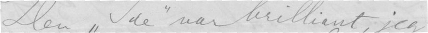Den Ide var brilliant; jeg
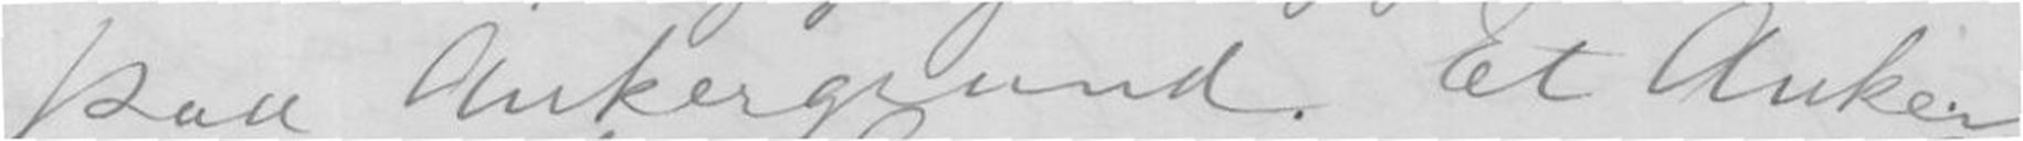paa Ankergrund. Et Anker
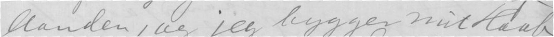Aanden, og jeg bygger mit Haab
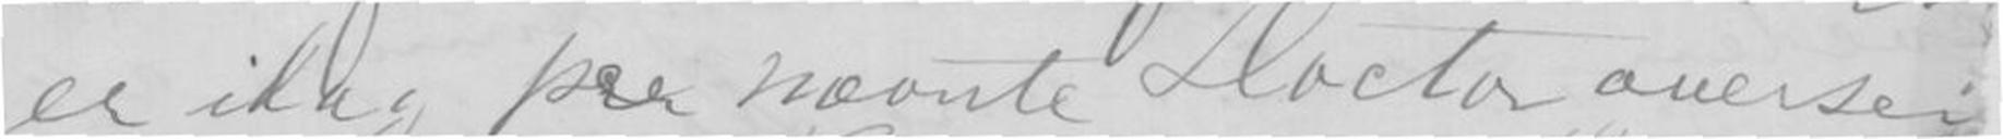er idag per nævnte Doctor oversen
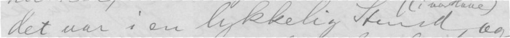det var i en lykkelig Stund , og
